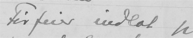Firfeier indlot jeg
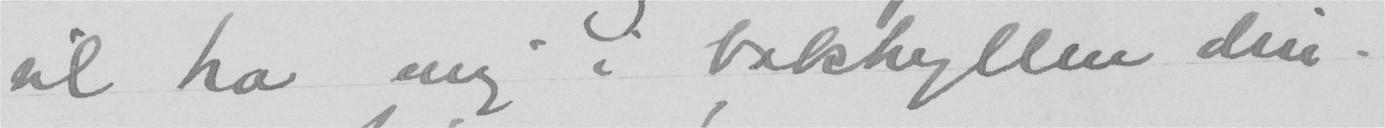vil ha mig i bokhyllen din.
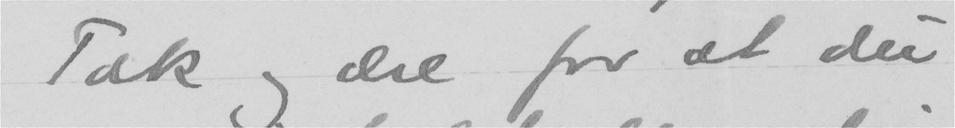Tak og ære for at du
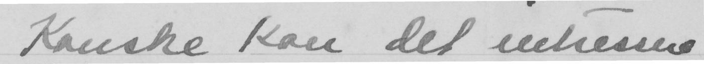Kanske kan det intressere
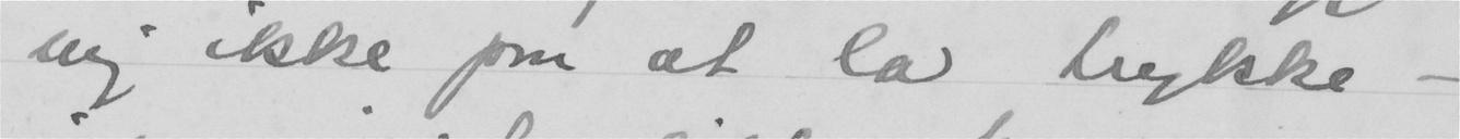mig ikke paa at la trykke -
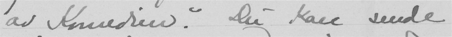av Komedien? Du kan sende
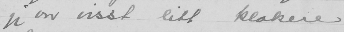jeg var visst litt klokere
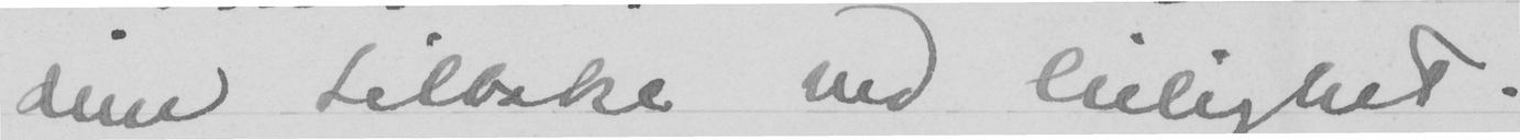dine tilbake ved leilighet.
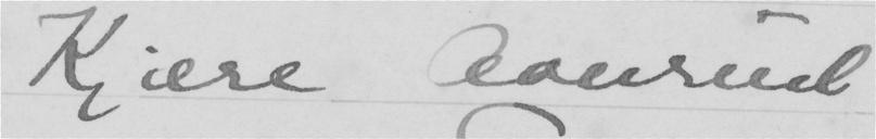Kjære Aanrud
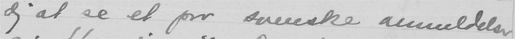dig at se et par svenske anmeldelser
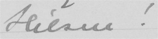Hilsen!
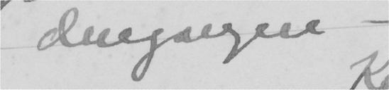dengangen -
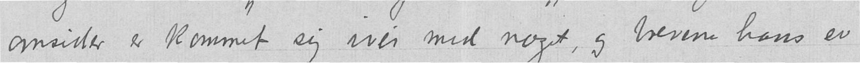omsider er kommet sig ivei med noget, og brevene hans er
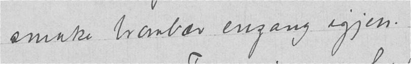smake brombær engang igjen.
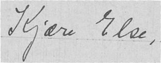Kjære Else,
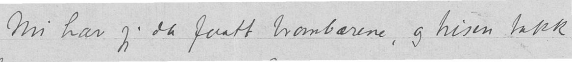Nu har jeg da faatt brombærene, og tusen takk
